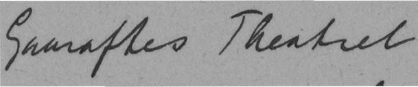Gaaraftes Theatret
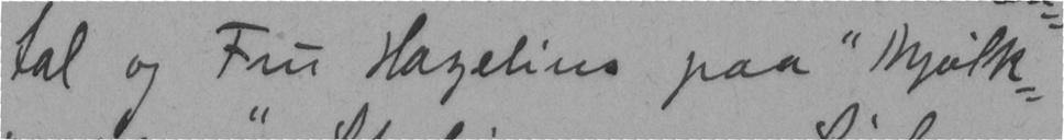tal og Fru Hagelius paa "Mjølk-
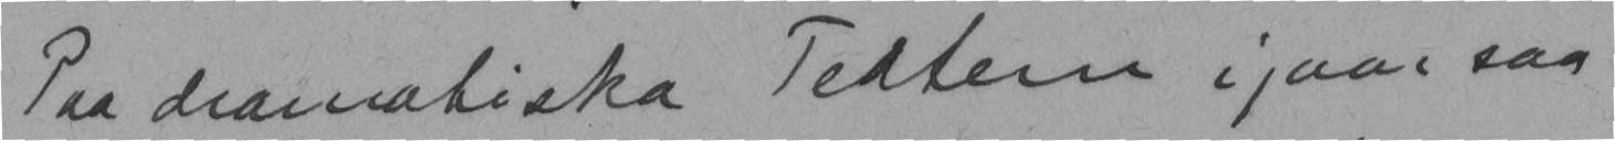Paa dramatiska Teatern igaar saa
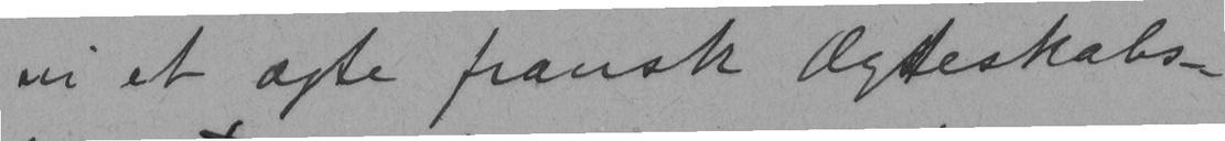vi et æfte fransk Ægteskabs-
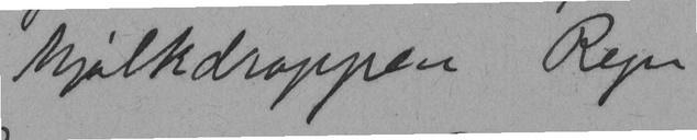Mjølkdroppen Regn
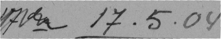17.5.04
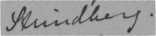Strindberg.
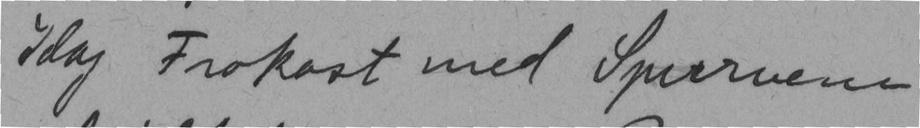Idag Frokast med Spurvene
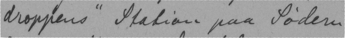droppens" Station paa Sødern
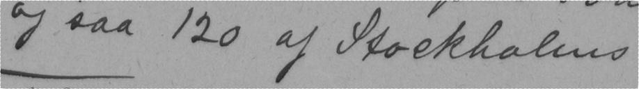og saa 120 af Stockholms
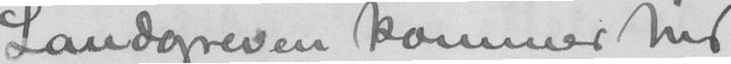Landgreven kommer hid
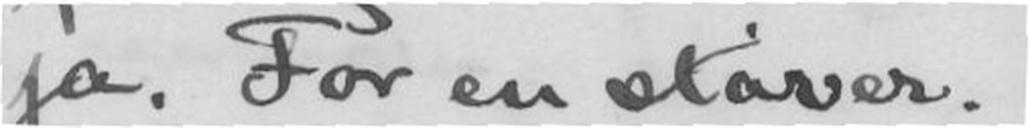ja. For en støver. Det har her
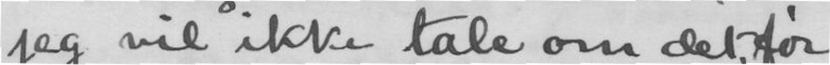jeg vil ikke tale om det, før
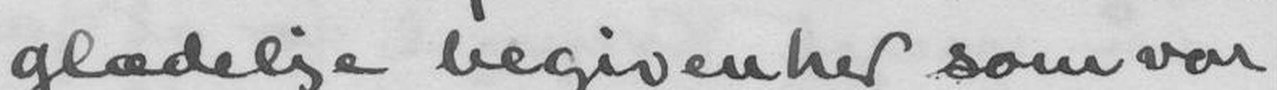glædelige begivenhed som var
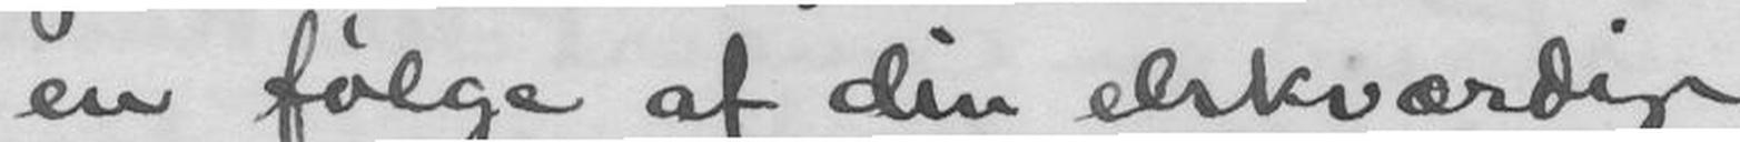en følge af din elskværdige
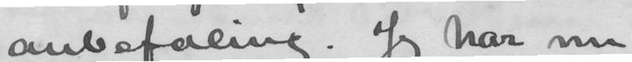anbefaling. Jeg har nu
